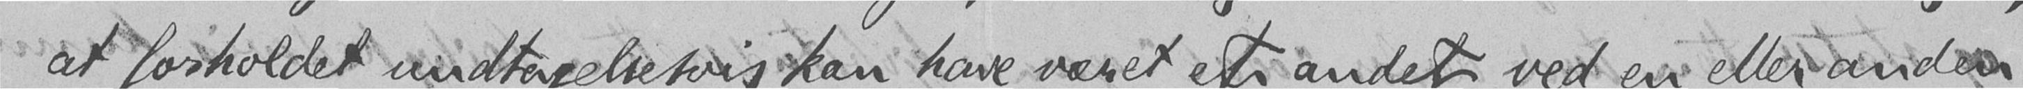at forholdet undtagelsesvis kan have været et andet ved en eller anden
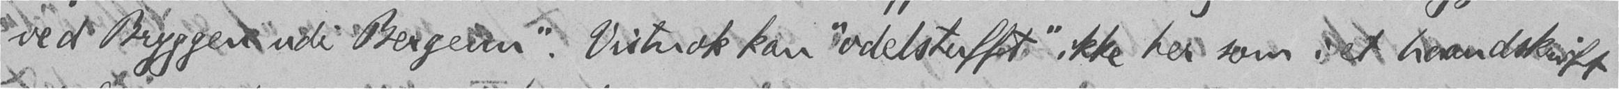ved Bryggen udi Bergenn". Vistnok kan "odelstufft" ikke her som i et haandskrift
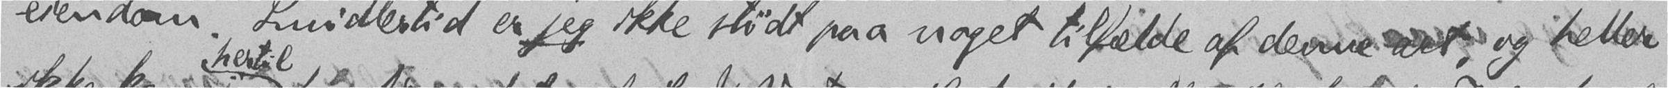eiendom. Imidlertid er jeg ikke stødt paa noget tilfælde af denne art, og heller
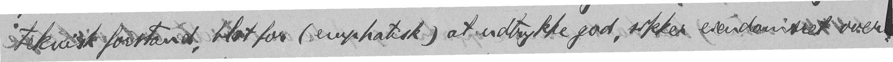teknisk forstand, blot for (emphatisk) at udtrykke god, sikker eiendomsret over-
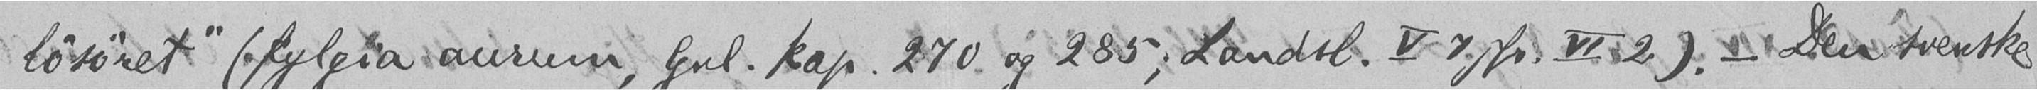løsøret" (fylgia aurum, Gul. kap. 270 og 285; Landsl. V 7 jfr. VI 2). - Den svenske
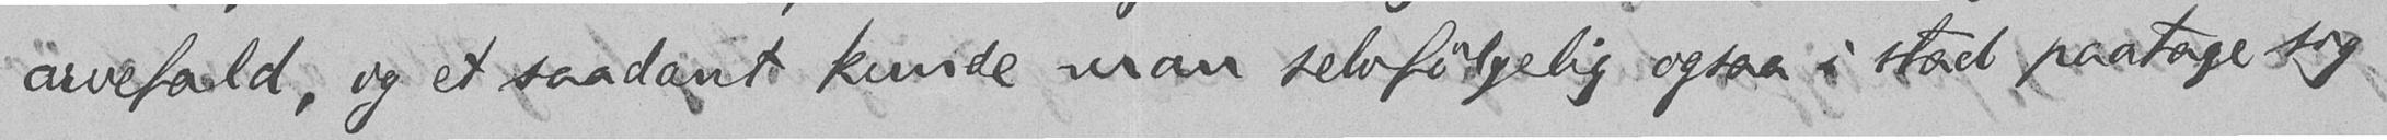arvefald, og et saadant kunde man selvfølgelig ogsaa i stad paatage sig
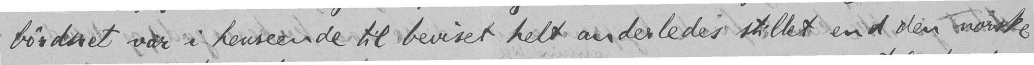bördsret var i henseende til beviset helt anderledes stillet end den norske
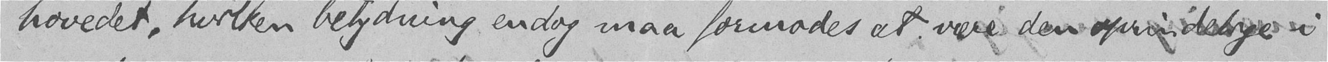hovedet, hvilken betydning endog maa formodes at være den oprindelige i
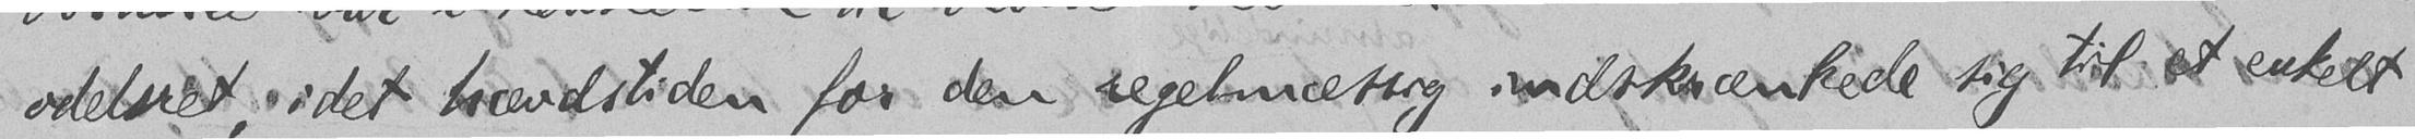odelsret, idet hævdstiden for den regelmæssig indskrænkede sig til et enkelt
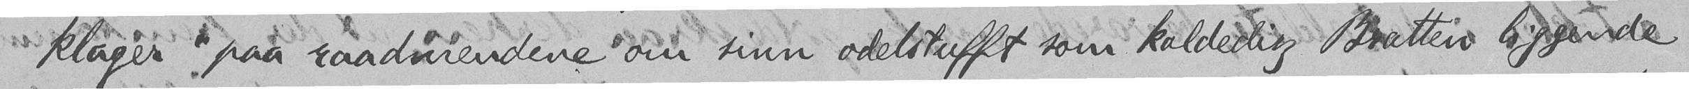klager "paa raadmendene om sinn odelstufft som kaldedig Bratten liggende
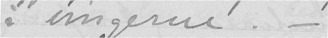i vingerne. -
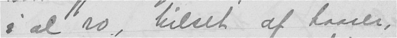i al ro, hilset af taarer,
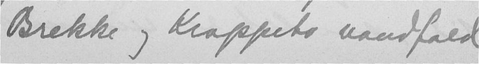Brekke og Krappeto vandfald.
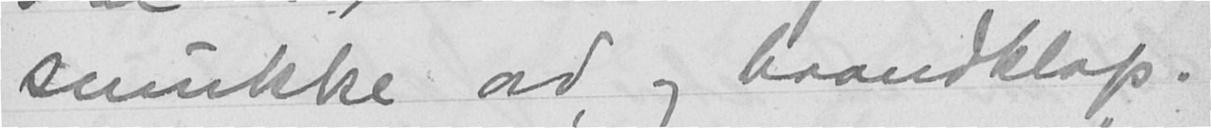smukke ord og haandklap.
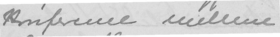konference mellem
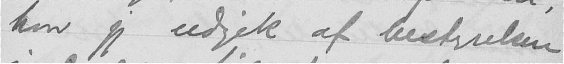hvor jeg udgik af bestyrelsen
